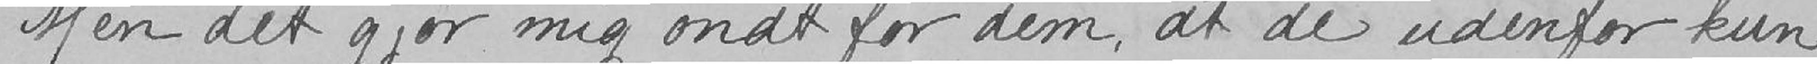Men det gjør mig ondt for dem, at de udenfor kun-
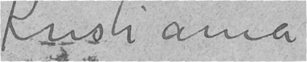Kristiania
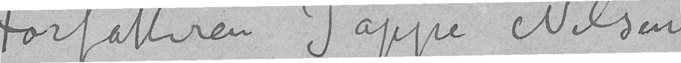Forfatteren Jappe Nilsen
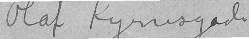Olaf Kyrresgade
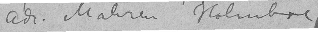Adr. Maleren Holmboe
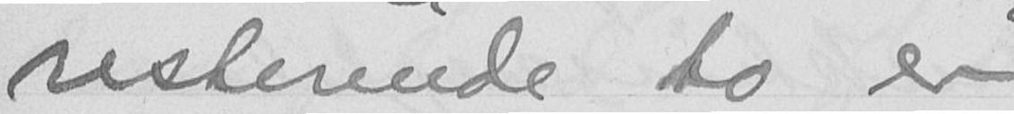resterende to er
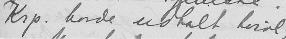Krp. havde udtalt tvivl,
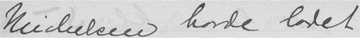Michelsen havde ladet
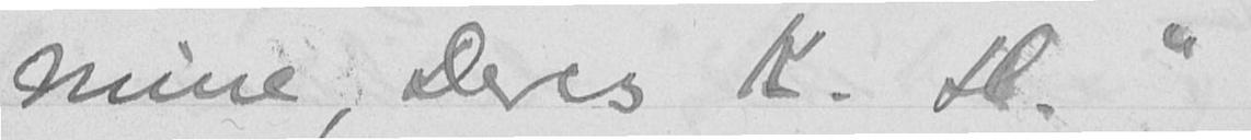mine, Deres K. H."
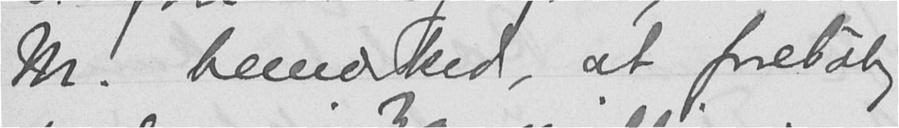M. bemærked, at foreløbig
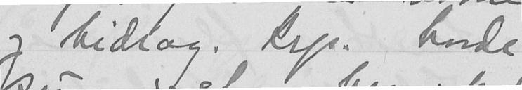og bidrag. Krp. havde
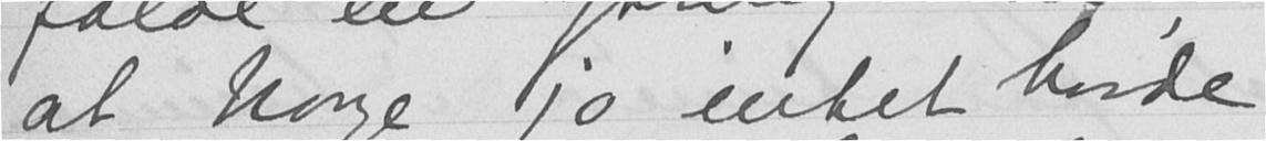at Norge jo intet havde
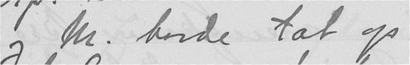og M. havde tat op
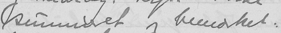summæret og bemærket:
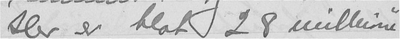"Her er blot 28 millione"
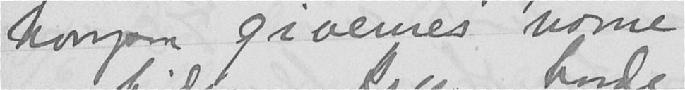hvorpaa givernes navne
